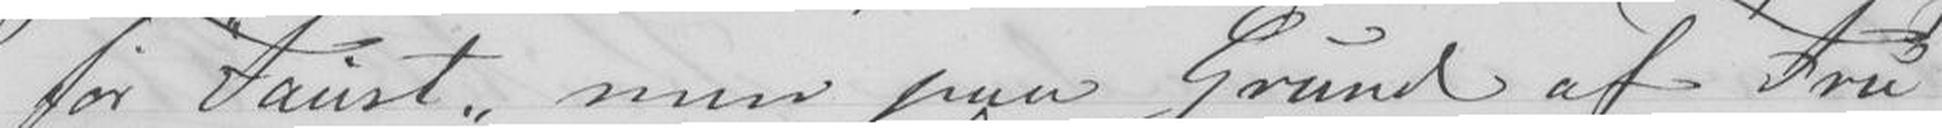for Faust men paa Grund af Fru
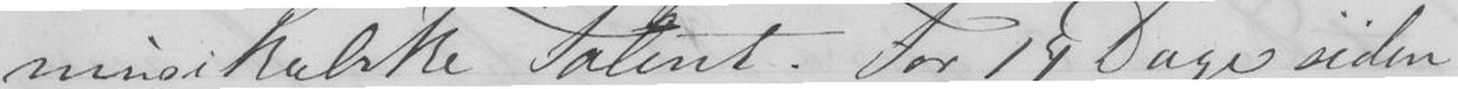musikalske Talent. For 14 Dage siden
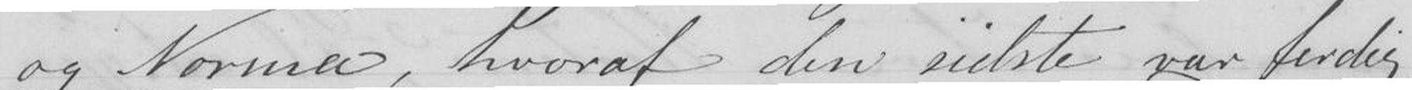og Norma, hvoraf den sidste var ferdig
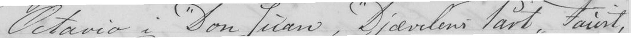Octavio i "Don Juan, Djævelens Part Faust,
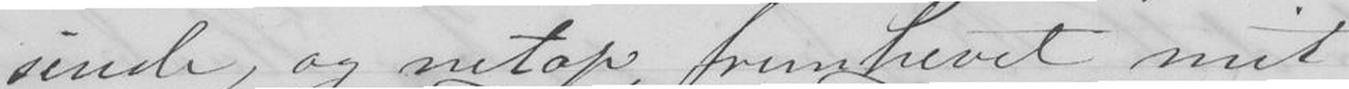sende, og netop fremhevet mit
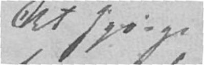At spørge
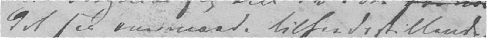det saa overmaade tilfredsstillende.
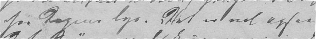for Dagens Lys. Det er vel ogsaa
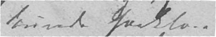kunde forklare
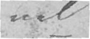vel
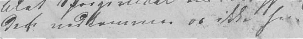det vedkommer os ikke her.
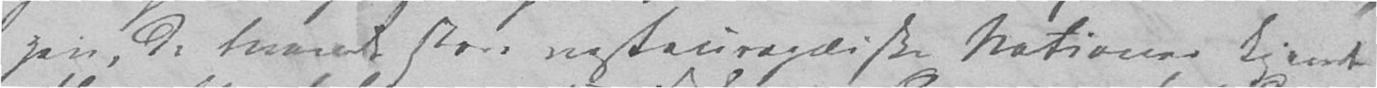gen, de tvende store vesteuropeiske Nationer kjende
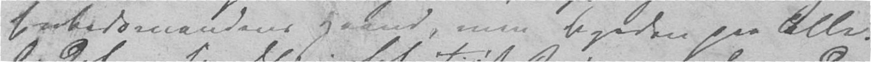Embedsmandens Haand, men Byrden paa Alle.
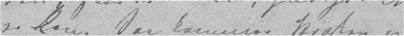er Lov. Saa kommer Præsten og
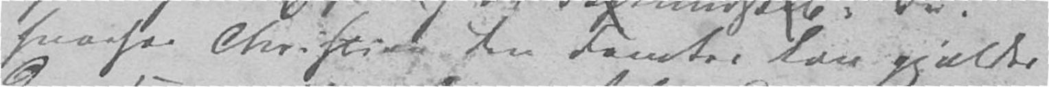hvorfor Christian den Femtes Lov gjælder
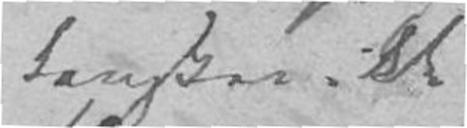kanskee ikke
